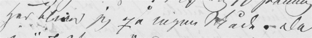Her bliver jeg på ingen Måde - da
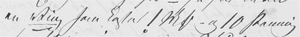en Ving som koster 1 Mf – og 10 Pennig.
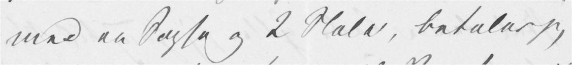med en Sopha og 2 Stole, betaler jeg
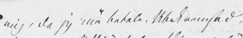mig, da jeg må betale. Ubekvemhed,
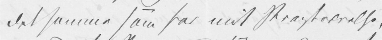det samme som for mit Pragtværelse,
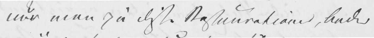når man på disse Restaurationer, beder
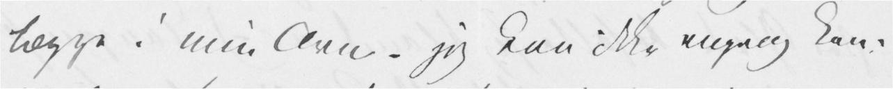lægge i min Ovn – jeg kan ikke engang kon-
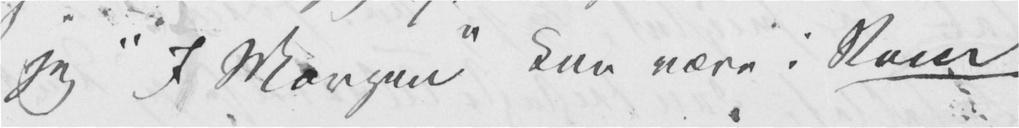jeg "I Morgen" kan være i Rom
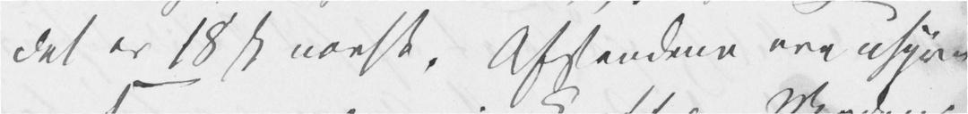det er 18 sk norsk. Afstandene ere uhyre
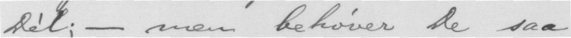Dél; - men behøver De saa
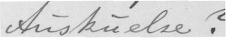Anskuelse?
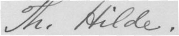Thi Hilde
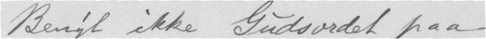Benyt ikke Gudsordet paa
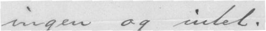ingen og intet.
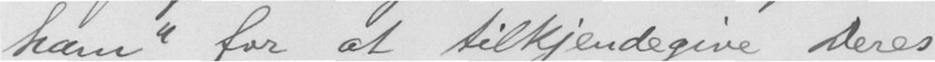ham" for at tilkjendegive Deres
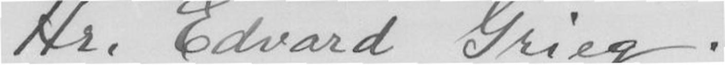Hr. Edvard Grieg
